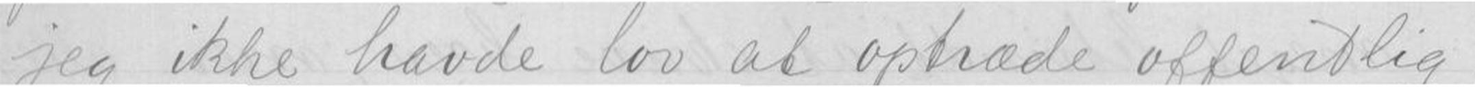jeg ikke havde lov at optræde offentlig,
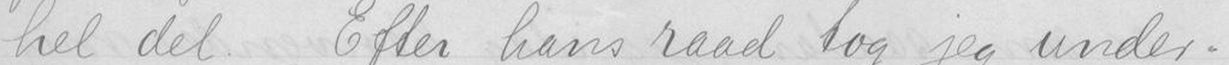hel del. Efter hans raad tog jeg under-
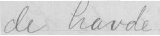de havde.
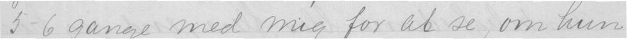5-6 gange med mig for at se om hun
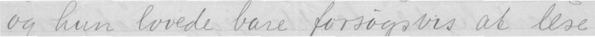og hun lovede bare forsøgsvis at lese
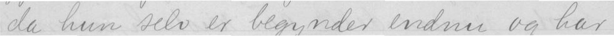da hun selv er begynder endnu og har
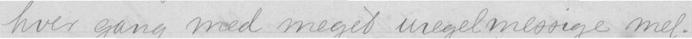hver gang med meget uregelmessige mel-
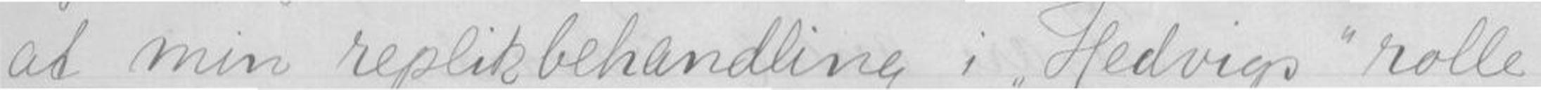at min replikbehandling i Hedvigs rolle
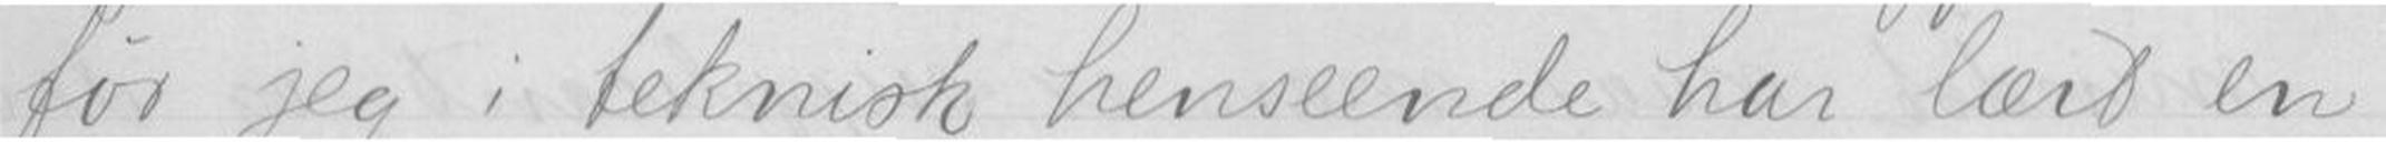før jeg i teknisk henseende har lært en
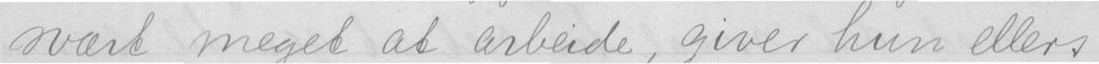svært meget at arbeide, giver hun ellers
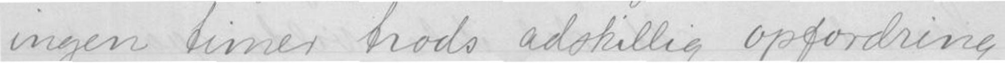ingen timer trods adskillig opfordring,
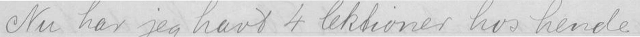Nu har jeg havt 4 lektioner hos hende
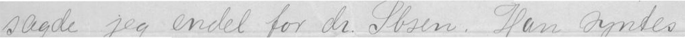sagde jeg endel for dr. Ibsen. Han syntes,
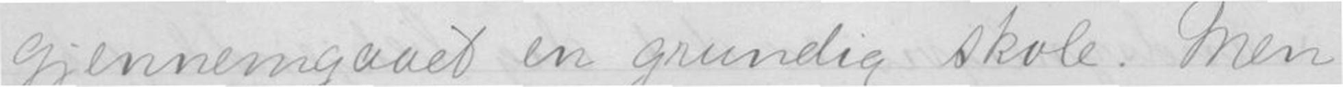gjennemgaaet en grundig skole. Men
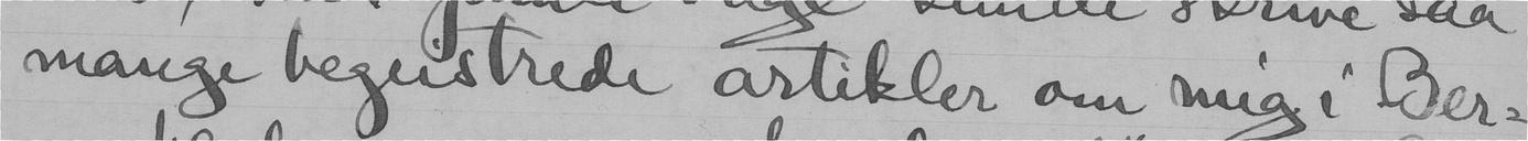mange begeistrede artikler om mig i Ber-
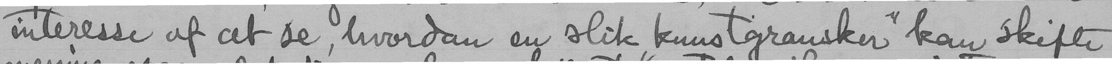interesse af at se, hvordan en slik "kunstgransker" kan skifte
